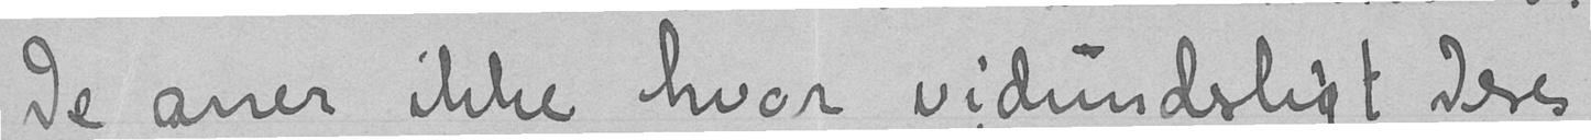De aner ikke hvor vidunderligt Deres
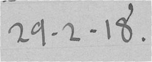29-2-18.
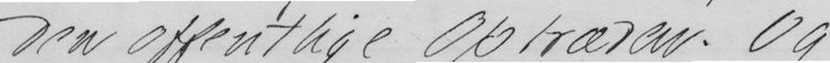den offentlige Optræden. og
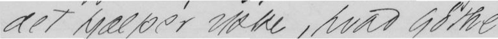det hjælper ikke, hvad
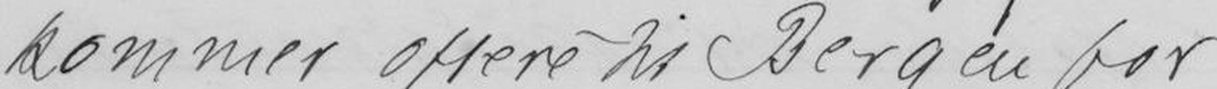kommer oftere til Bergen for
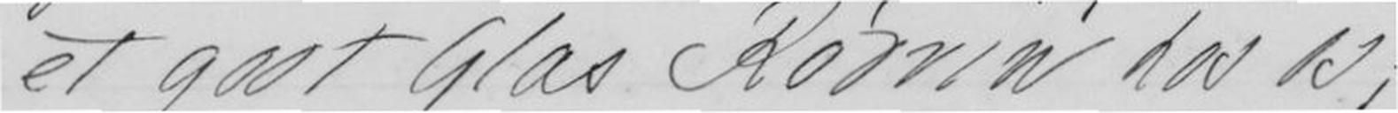et godt glas Rødvin hos os;
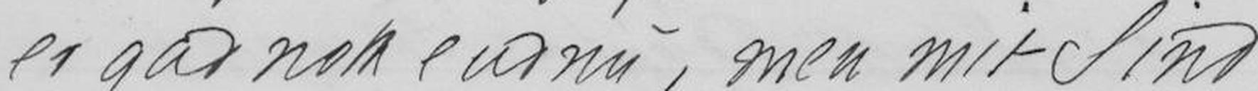er god nok endnu, men mit Sind
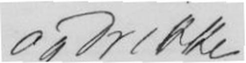og drikke
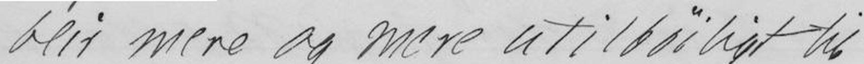blir mere og mere utilbøiligt til
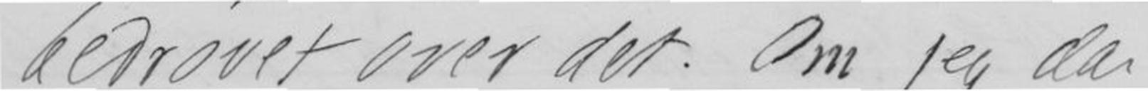bedrøvet over det. Om jeg da
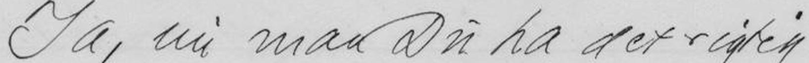Ja, nu maa Du ha det rigtig
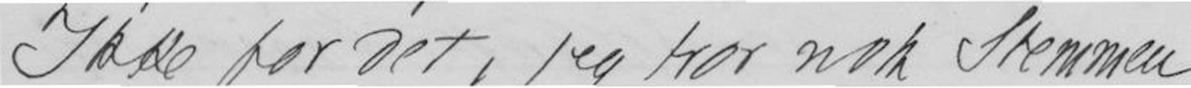Ikke for det, jeg tror nok Stemmen
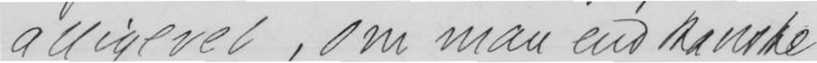alligevel, om man end kanske
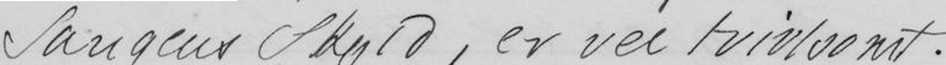Sangens skyld, er vel tvivlsomt.
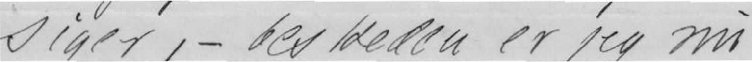siger,- beskeden er jeg nu
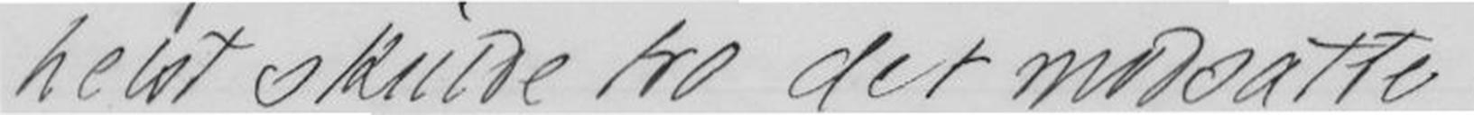helst skulde tro det modsatte.
